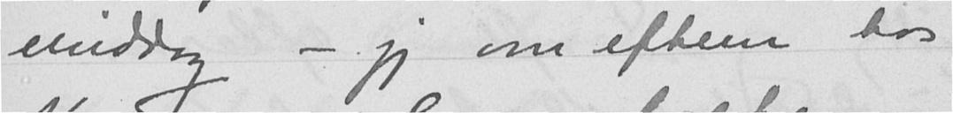middag - jeg om efterm hos
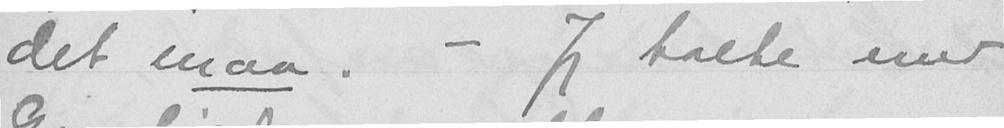det maa. - Jeg talte med
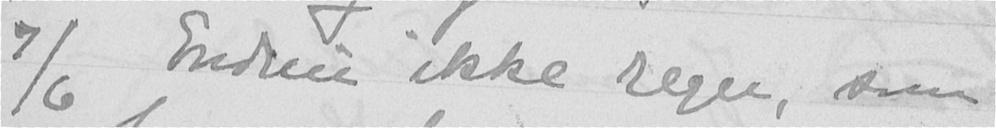7/6 Endnu ikke regn, som
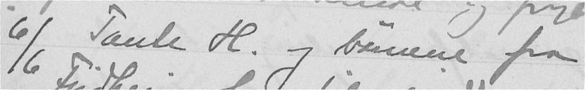6/6 Tante H. og børnene fra
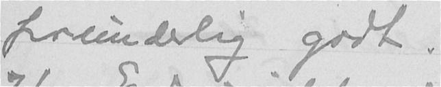forunderlig godt.
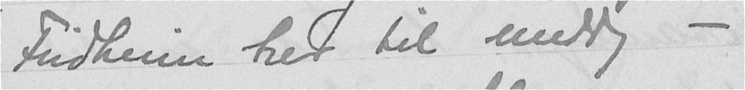Fridheim her til middag -
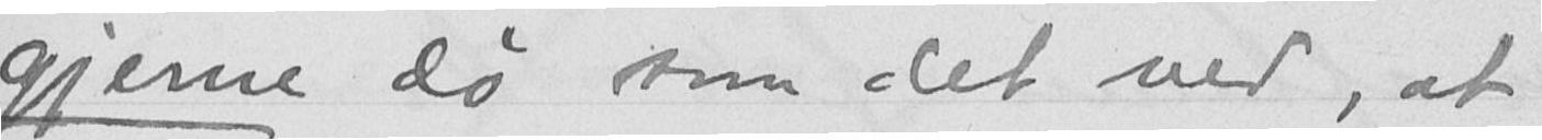gjerne dø som det ved, at
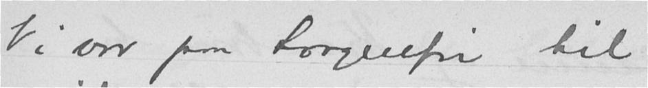Vi var paa Sorgenfri til
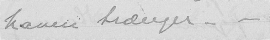haven trænger. -
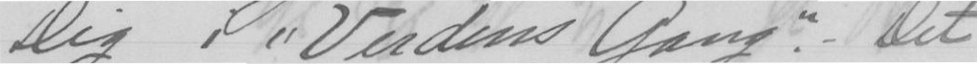Dig i "Verdens Gang". - Det
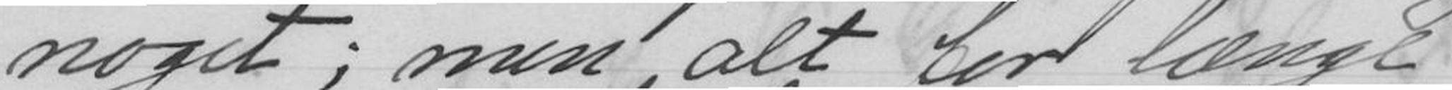noget; men alt for længe
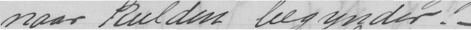naar kulden begynder. -
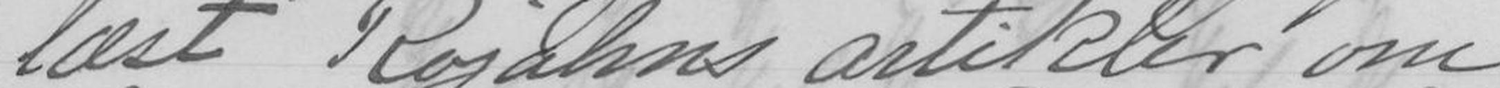læst Rojahns artikler om
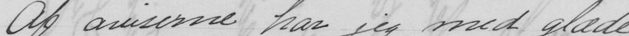Af aviserne har jeg med glæde
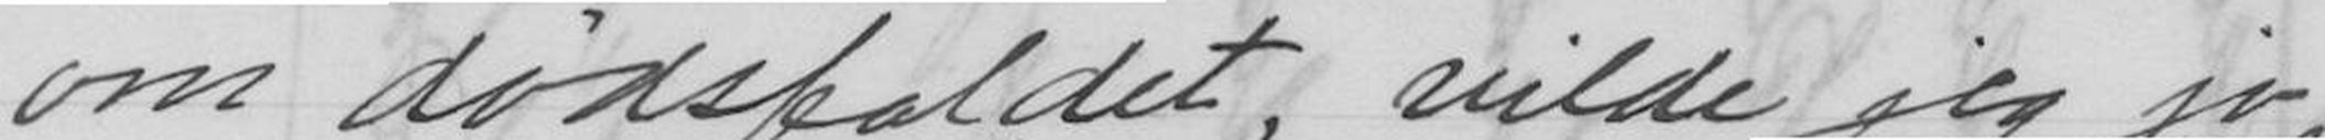om dødsfaldet, vilde jeg jo
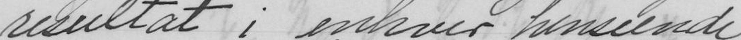resultat i enhver henseende.
